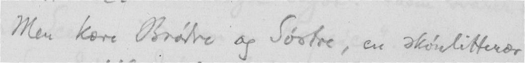Men kære Brødre og Søstre, en skønlitterær
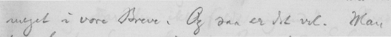meget i vore Breve. Og saa er det vel. Man
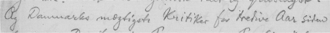Og Danmarks mægtigste Kritiker for tredive Aar siden
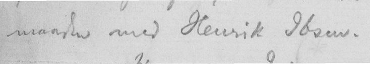maaden med Henrik Ibsen.
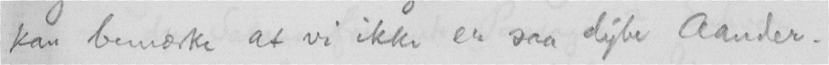kan bemærke at vi ikke er saa dybe Aander.
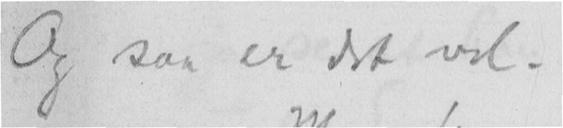Og saa er det vel.
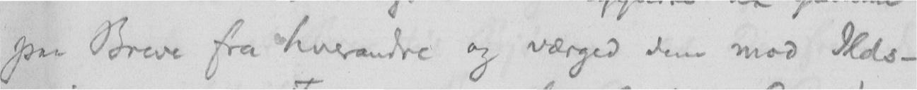paa Breve fra hverandre og værged dem mod Ilds-
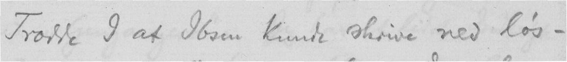Trodde I at Ibsen kunde skrive ned løs-
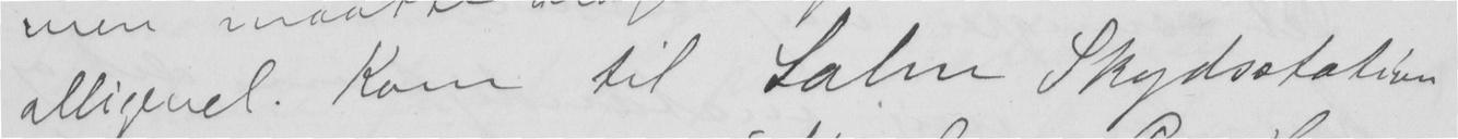alligevel. Kom til Lalm Skydsstation
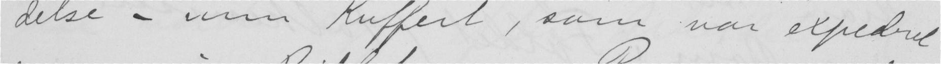delse - min Kuffert, som var expederet
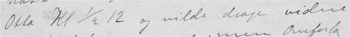Otta Kl 1½ 12 og vilde drage videre
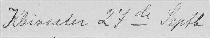Kleivsæter 27de Septb
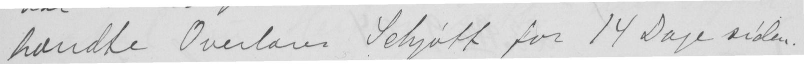hændte Overlærer Schjøtt for 14 Dage siden.
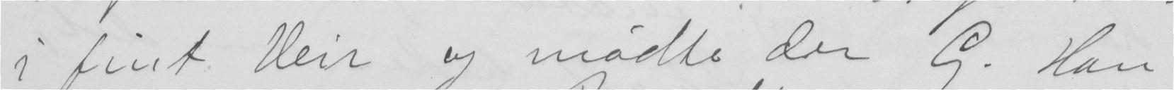i fint Veir og mødte der G. Han
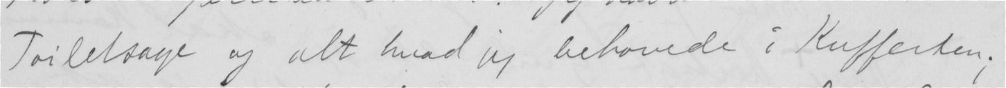Toiletsage og alt hvad jeg behøvede i Kufferten,
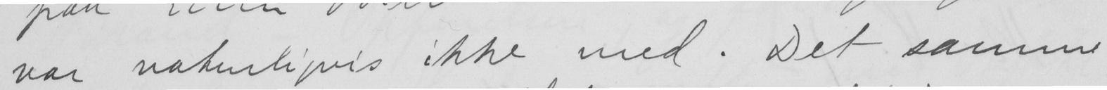var naturligvis ikke med. Det samme
